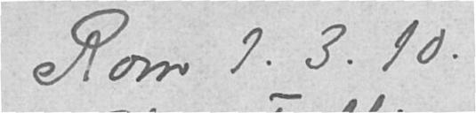Rom 1.3.10.
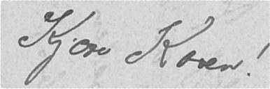Kjære Karen!
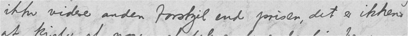ikke videre anden forskjel end prisen, det er ikkeno
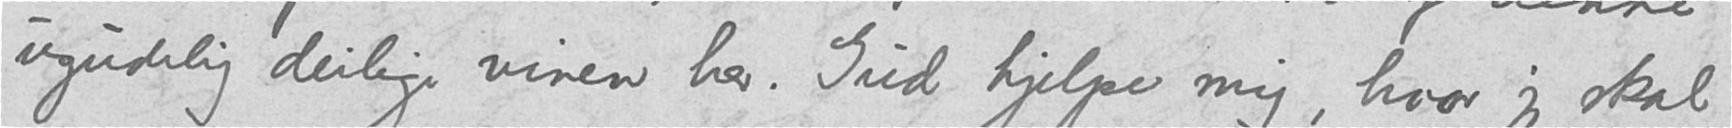ugudelig deilige vinen her. Gud hjelpe mig, hvor jeg skal
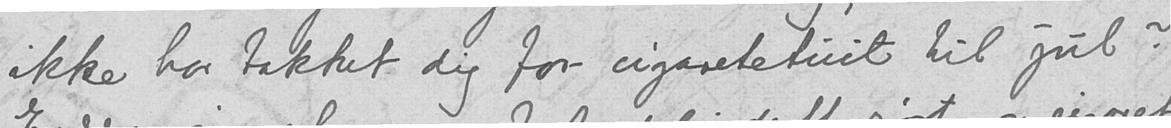ikke har takket dig for cigaretetuiet til jul?
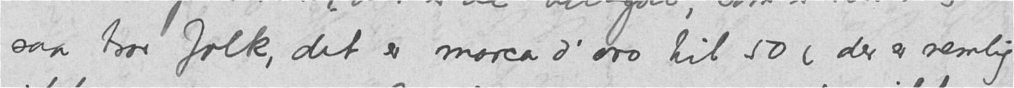saa tror folk, det er morca d'oro til 50 (der er nemlig
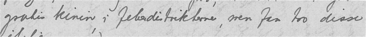gratis kinin i feberdistrikterne, men fan tro disse
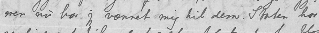men nu har jeg vænnet mig til dem. Staten har
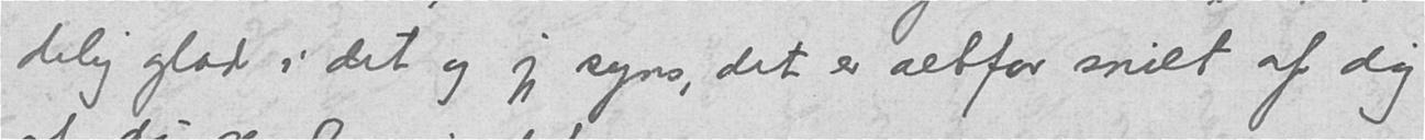delig glad i det og jeg syns, det er altfor snilt af dig
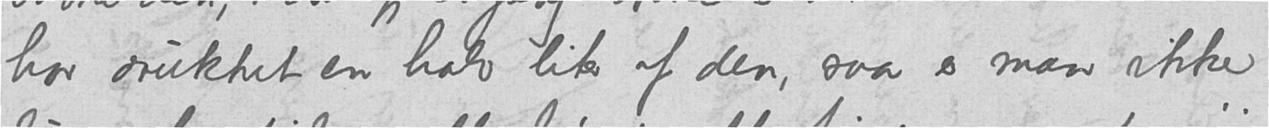har drukket en halv liter af den, saa er man ikke
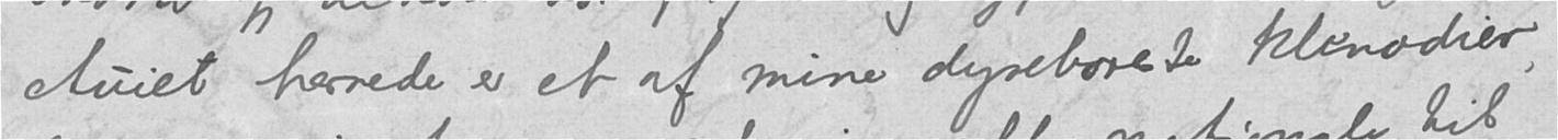etuiet hernede er et af mine dyrebareste klenodier,
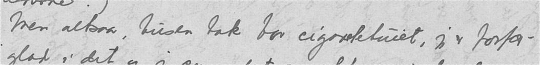Men altsaa tusen tak for cigaretetuiet, jeg er forfær-
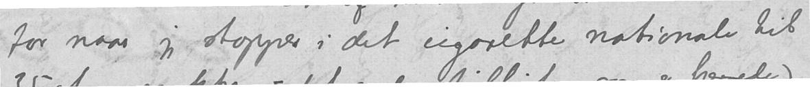for naar jeg stopper i det cigarette nationale til
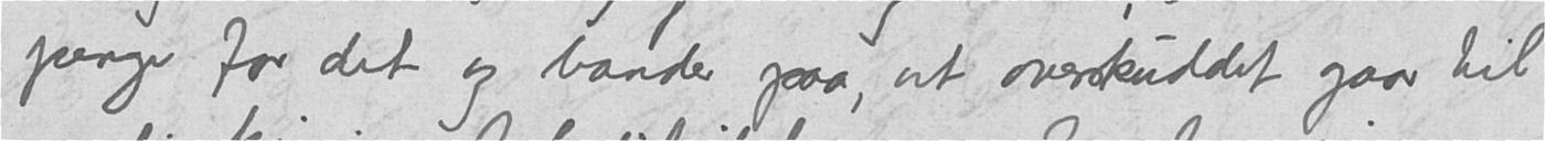penge for det og bander paa, at overskuddet gaar til
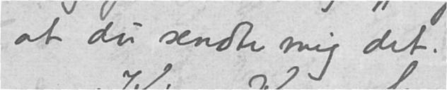at du sendte mig det.
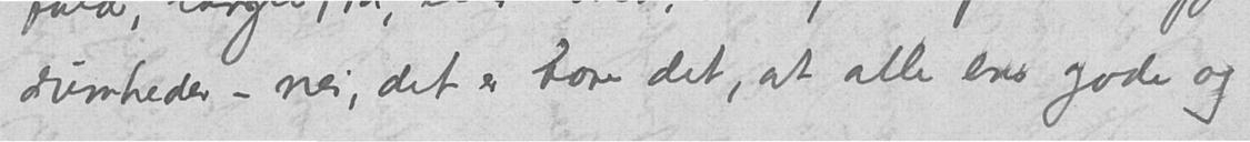dumheder – nei, det er bare det, at alle ens gode og
